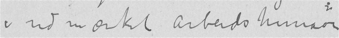i udmærket Arbeidshumør
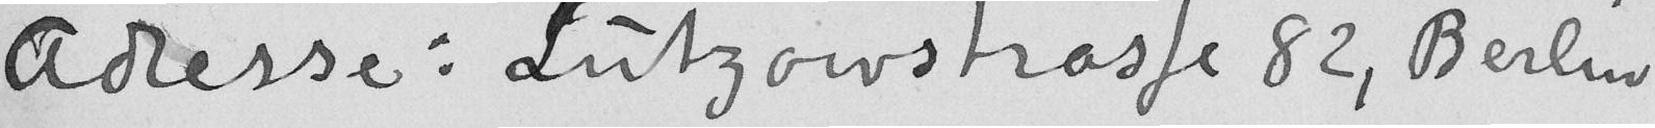Adresse: Lützowstrasse 82, Berlin
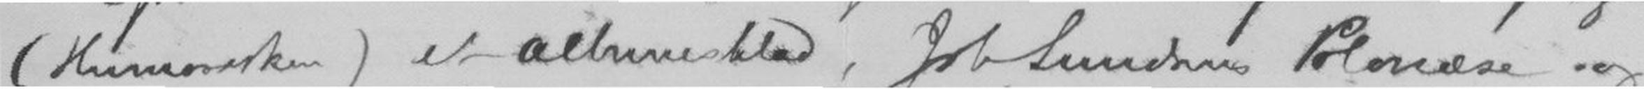(Humoresken) et albumblad Joh Lundens Polonæse og
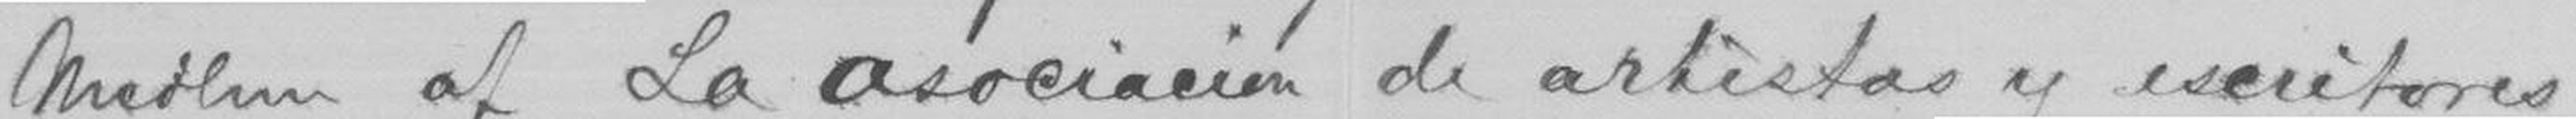medlem af La Asociacien de artistas og escritores
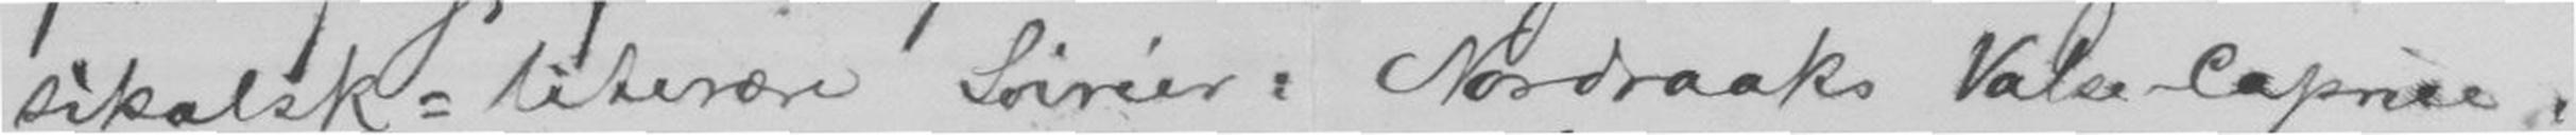sikalsk=Literære Soiroer: Nordraaks Valse-Caprice,
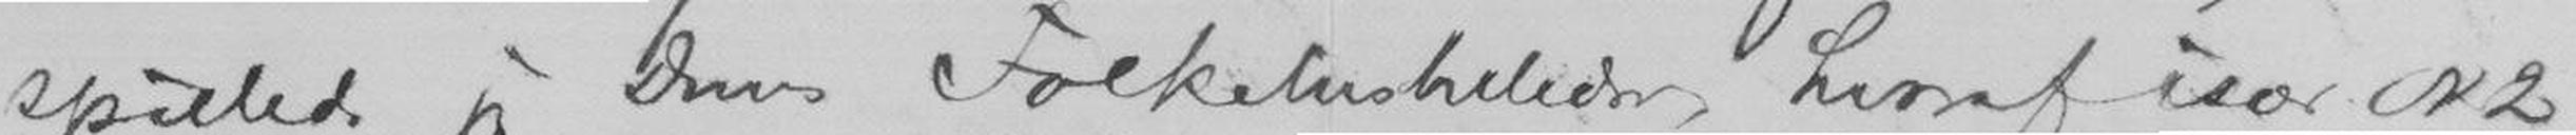spilled jeg Deres Folketersbilleder, hvoraf især N 2
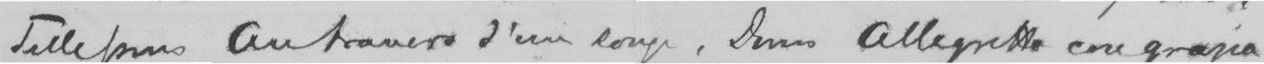Tellefsens Autrovers d'un songe, Deres Allegretto con grazia
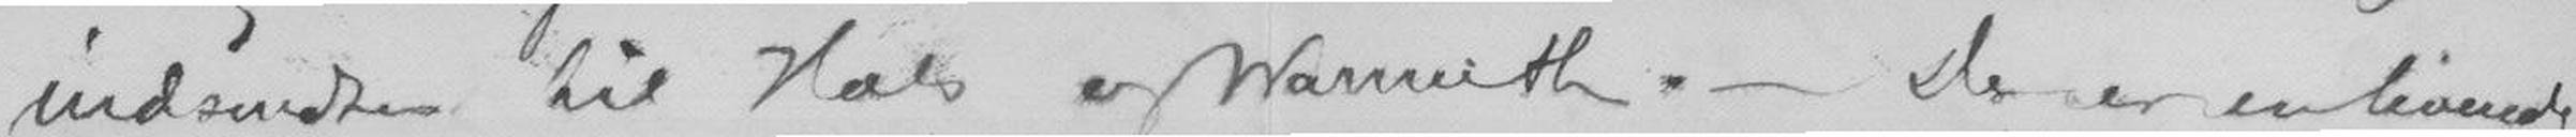indsændtes til Hals og Warmuth. - De er en livende
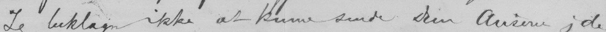Jeg beklager ikke at kunne sende Dem Aviserne; de
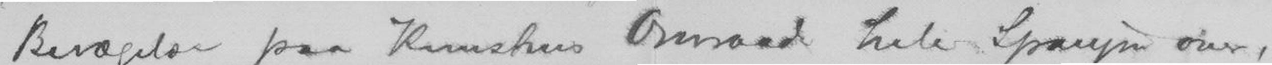Bevægelse paa Kunstens Omraade hele Spanjen over,
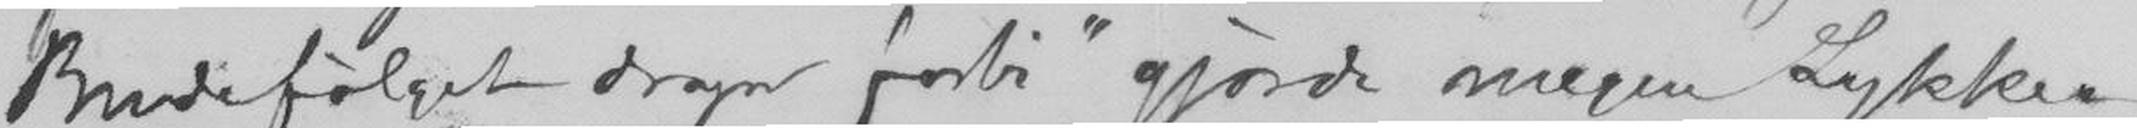"Brudefølget drager forbi" gjorde megen Lykke. -
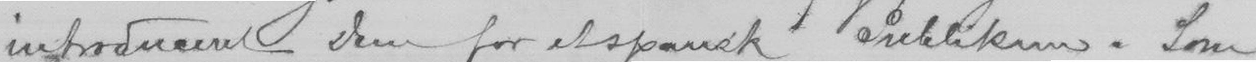introduceret Dem for etspansk Publikum. Som
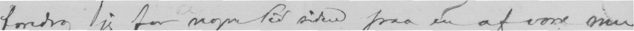foredro, jeg for nogen tid siden paa en af vore mu-
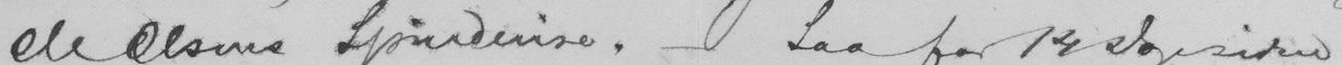Ole Olsens Spindevise. - Saa for 14 Dage siden
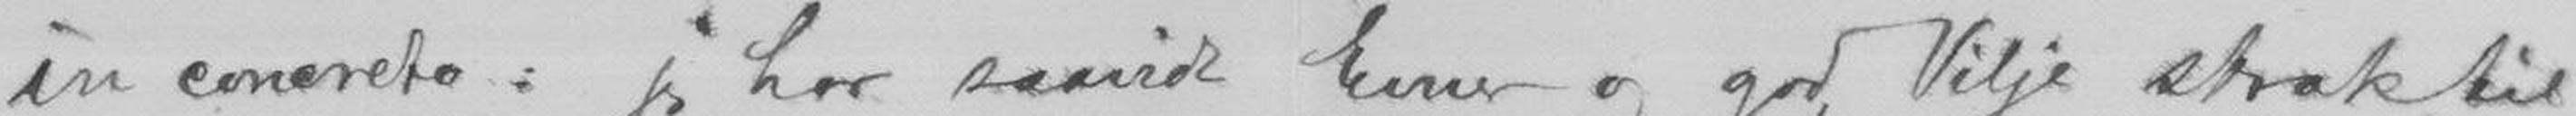in concreto: jeg har saavidt Evner og god Vilje strak til
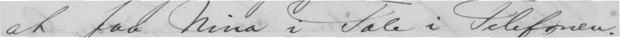at faa Nina i Tale i Telefonen.
